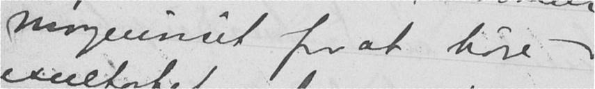morgenvisit for at høre
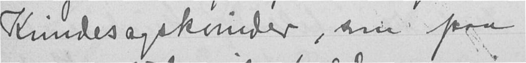Kvindesagskvinder, som paa
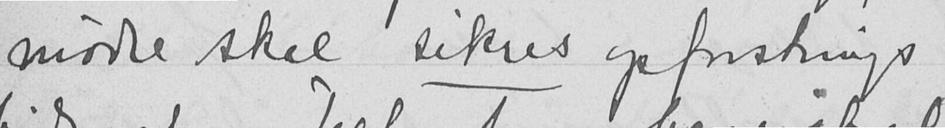mødre skal sikres opfostrings
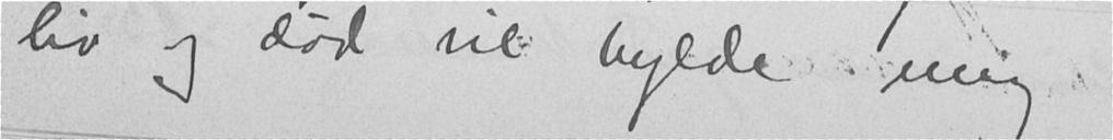liv og død vil hylde mig
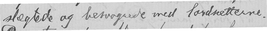slægtede og besvogrede med lordsætterne.
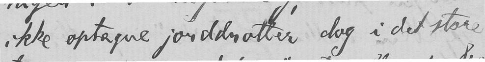ikke optagne jorddrotter dog i det store
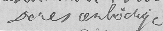Deres ærbødige
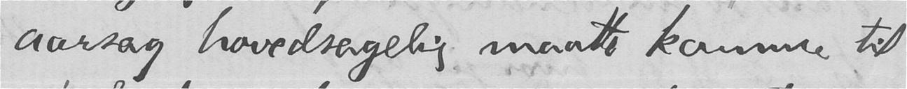aarsag hovedsagelig maatte komme til
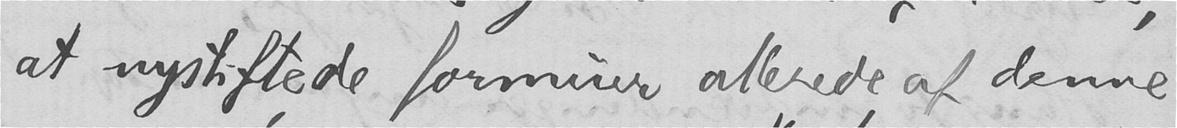at nystiftede formuer allerede af denne
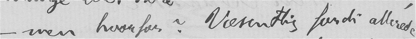- men hvorfor? Væsentlig fordi allerede
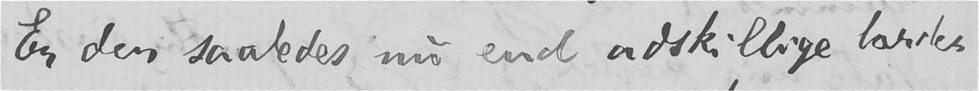Er der saaledes nu end adskillige lorder
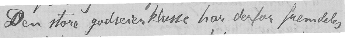Den store godseierklasse har derfor fremdeles,
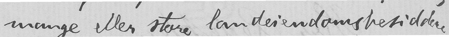mange eller store landeiendomsbesiddere,
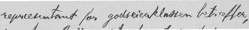repræsentant for godseierklassen betræffer,
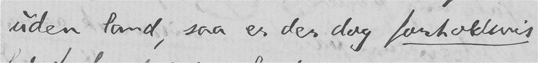uden land, saa er der dog forholdsvis
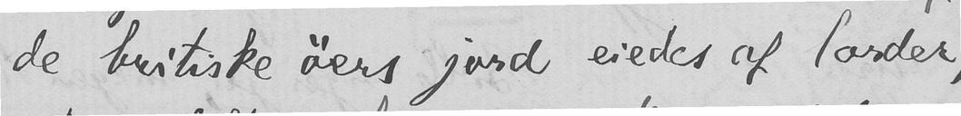de britiske øers jord eiedes af lorder,
